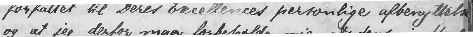forfattet til Deres Excellences personlige afbenyttelse,
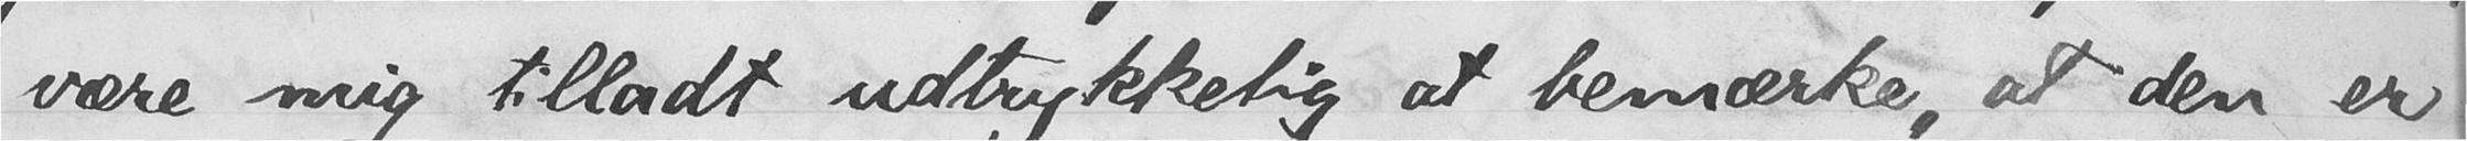være mig tilladt udtrykkelig at bemærke, at den er
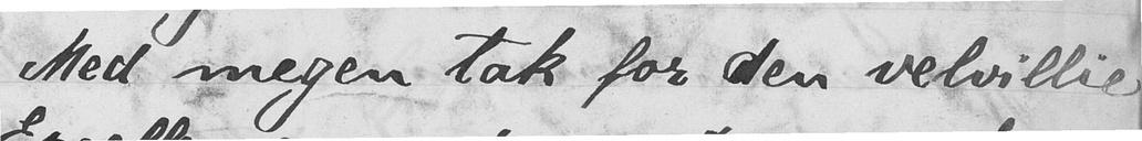Med megen tak for den velvillie,
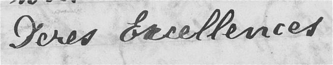Deres Excellences
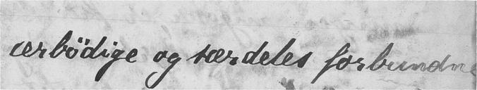ærbødige og særdeles forbundne
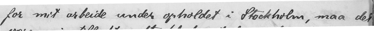for mit arbeide under opholdet i Stockholm, maa det
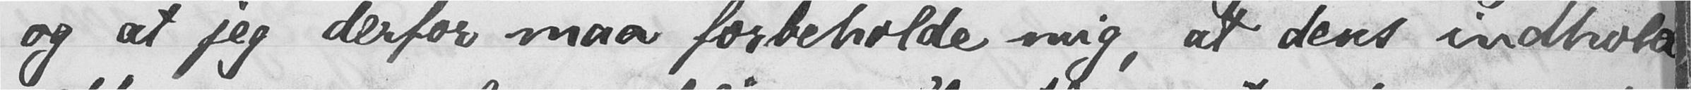og at jeg derfor maa forbeholde mig, at dens indhold
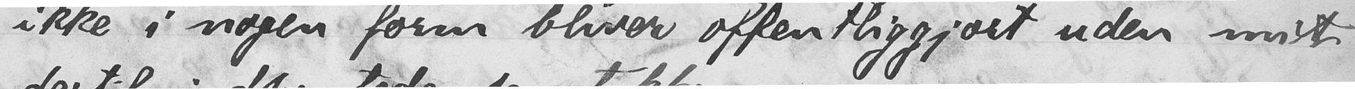ikke i nogen form bliver offentliggjort uden mit
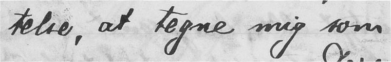telse, at tegne mig som
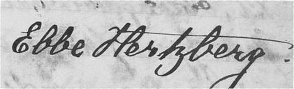Ebbe Hertzberg.
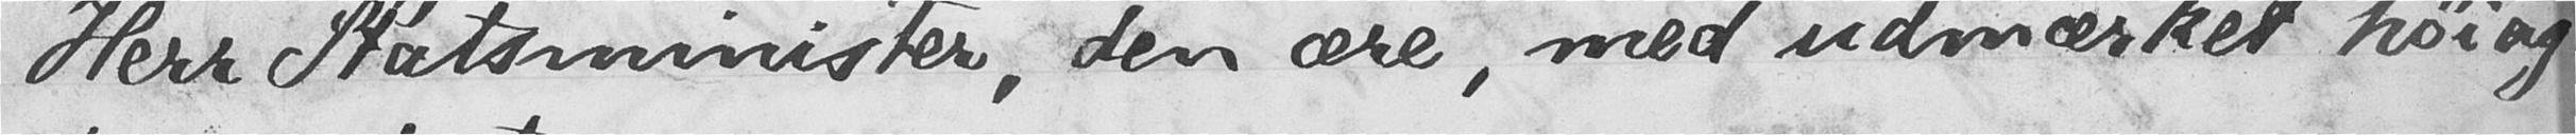Herr Statsminister, den ære, med udmærket høiag-
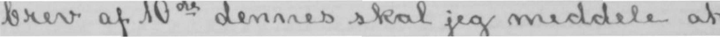brev af 10de dennes skal jeg meddele at
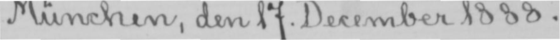München, den 17. December 1888.
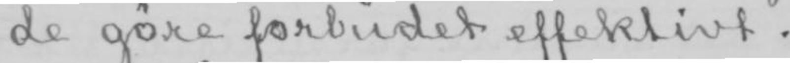de gøre forbudet effektivt.
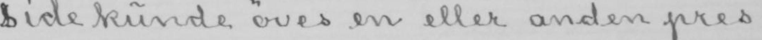side kunde øves en eller anden pres-
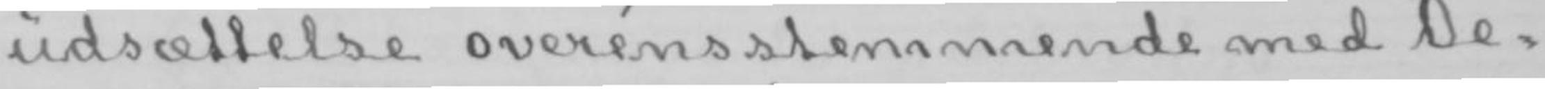udsættelse overénsstemmende med De-
